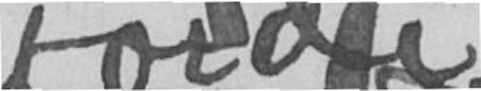fordi
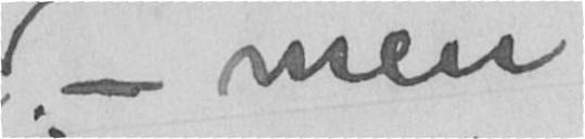- men
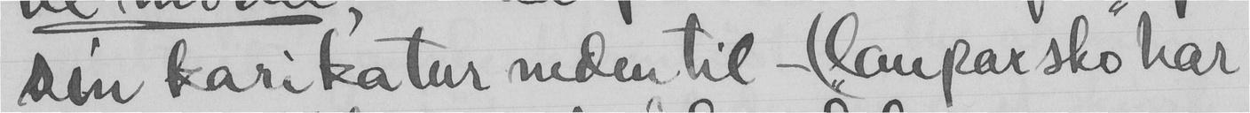sin karikatur nedentil - ("lauparsko" har
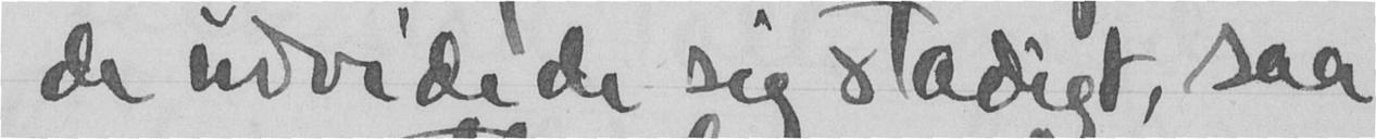de udvidede sig stadigt, saa
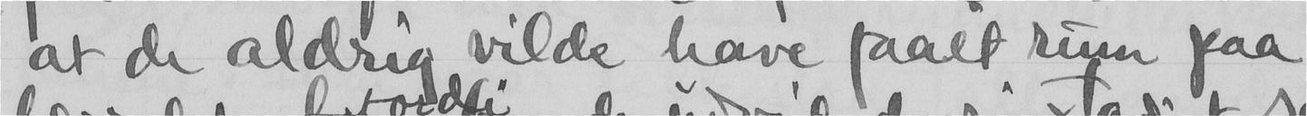at de aldrig vilde have faaet rum paa
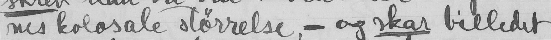nes kolosale størrelse, - og skar billedet
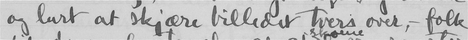og lurt at skjære billedet tvers over, - folk
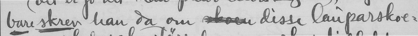bare skrev han da om  disse lauparskoe-
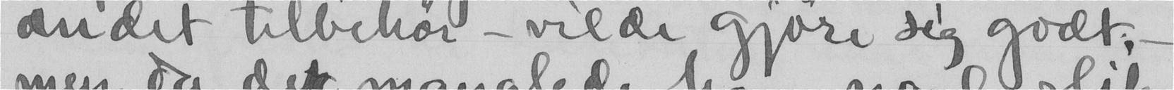andet tilbehør - vilde gjøre sig godt, -
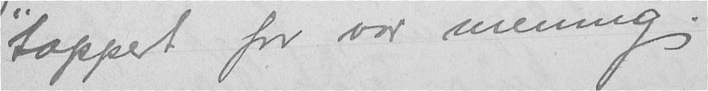tappert for vor mening.
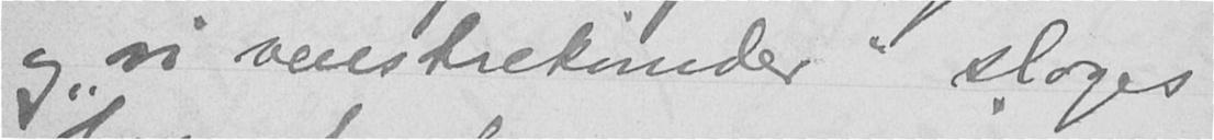og "vi venstrekvinder" sloges
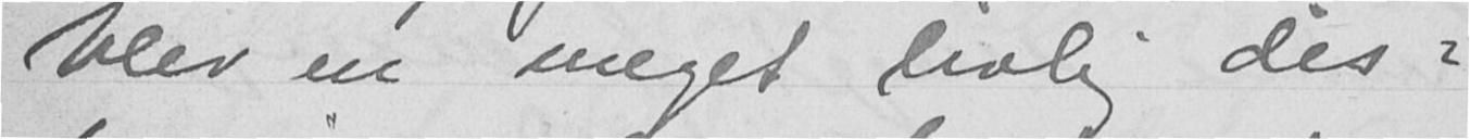blev en meget livlig dis-
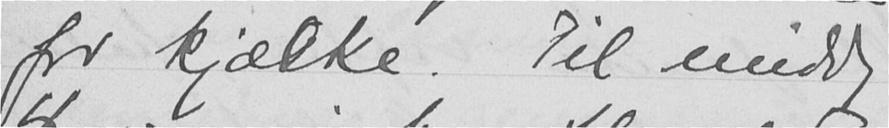for kjælke. Til middag
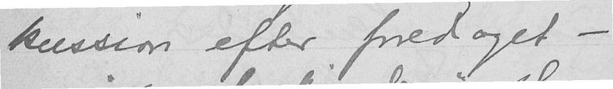kussion efter foredaget -
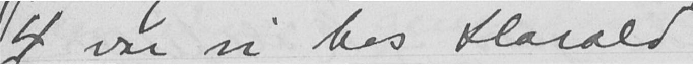4 var vi hos Harald
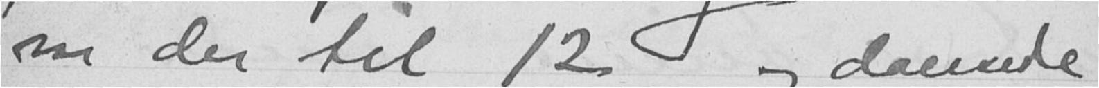var der til 12 og dansede
